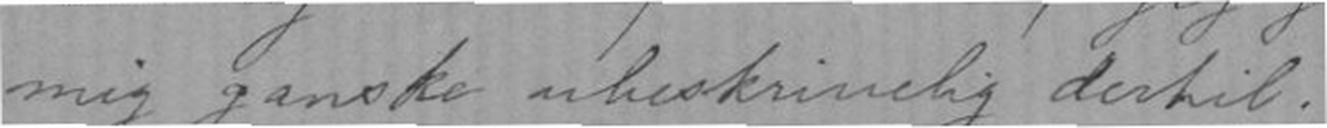mig ganske ubeskrivelig dertil.
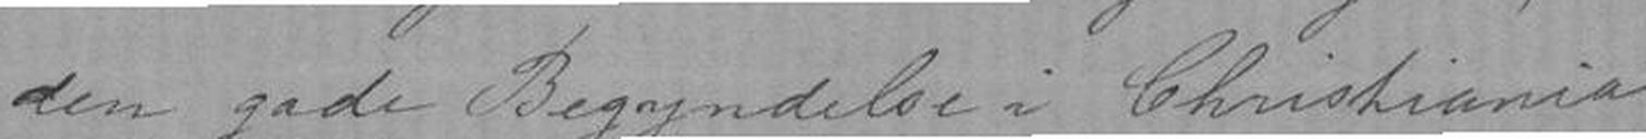den gode Begyndelse i Christiania
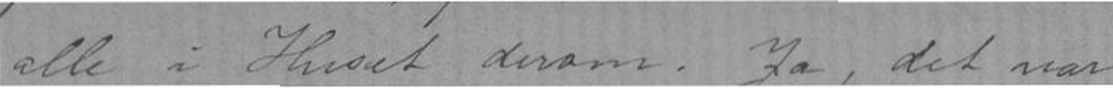alle i Huset derom. Ja, det var
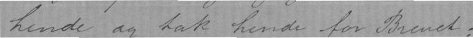hende og tak hende for Brevet;
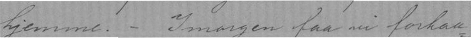hjemme. - Imorgen faa forhaa
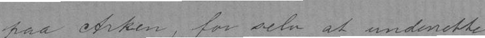paa Arken, for selv at underrette
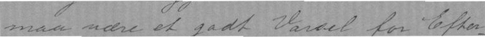maa være et godt Varsel for Efter-
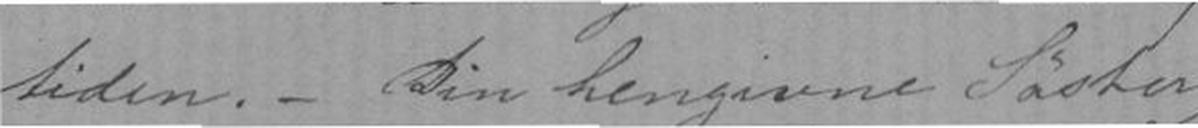tiden. - Din hengivne Søster
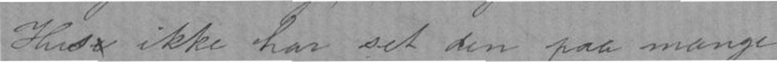Huse ikke har set den paa mange
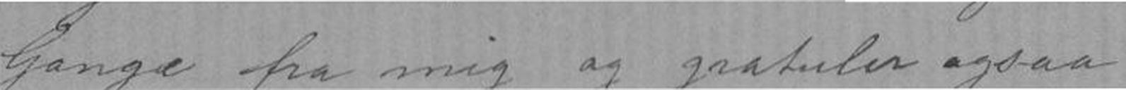Gange fra mig og gratuler ogsaa
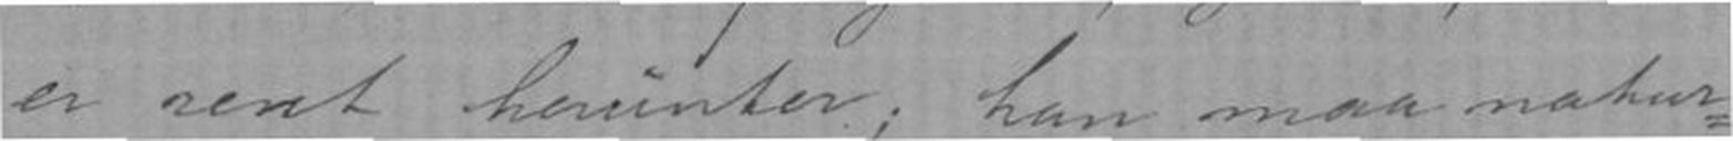er rent herünter; han maa nartur-
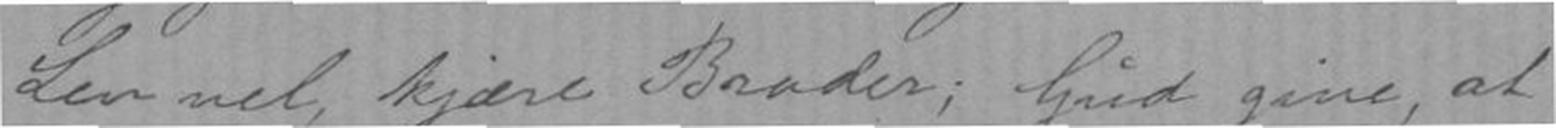Lev vel, kjære Broder; Gud give, at
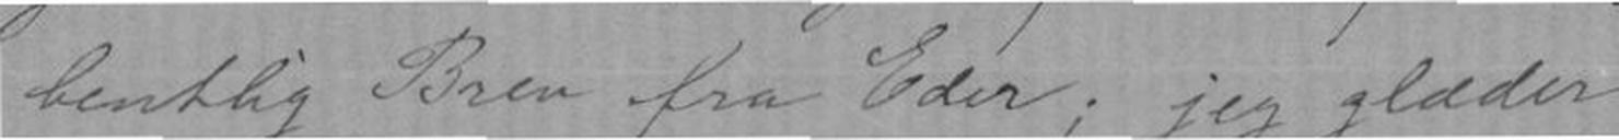bentlig Brev fra Eder; jeg glæder
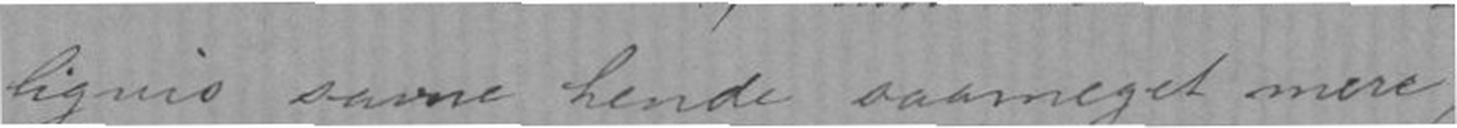ligvis savne hende saameget mere,
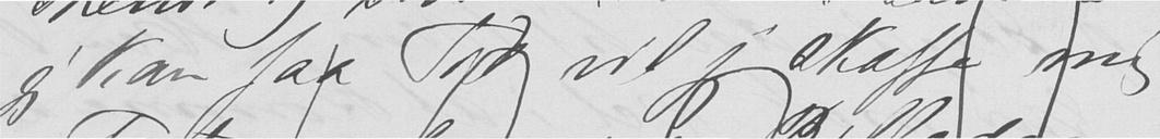jeg kan faa Tid vil jeg skaffe mig
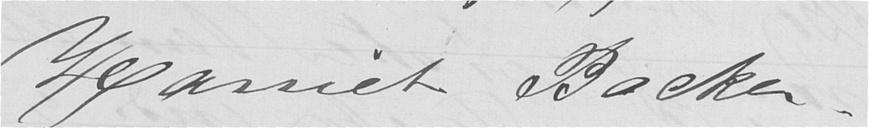Harriet Backer.
